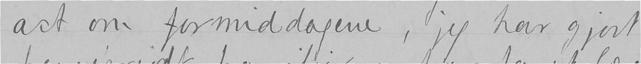act om formiddagene, jeg har gjort
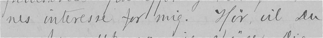nes interesse for mig. Hør, vil Du
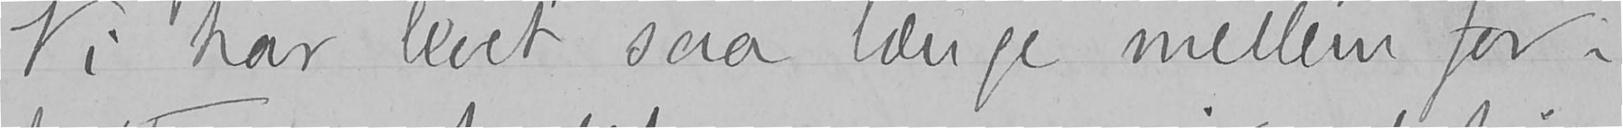Vi har levet saa længe mellem for-
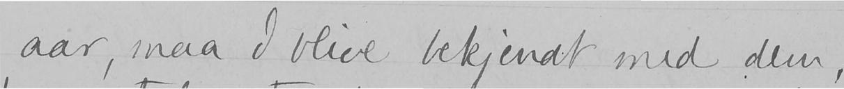aar, maa I blive bekjendt med dem,
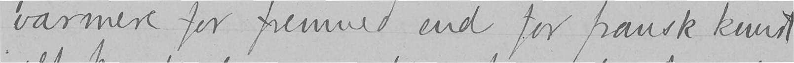varmere for fremmed end for fransk kunst
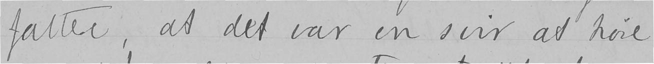fattere, at det var en seir at høre
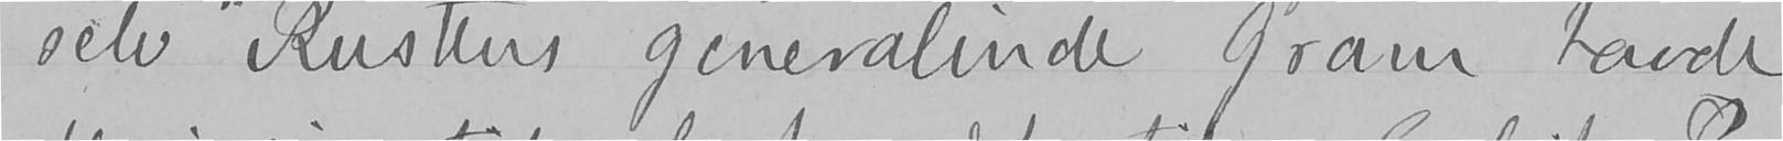selv Rustens generalinde Gram havde
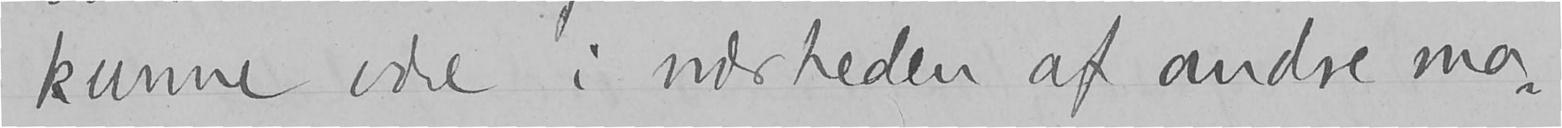kunne være i nærheden af andre ma-
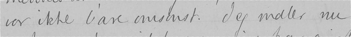var ikke bare omsinst. Jeg maler nu
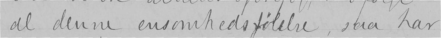al denne ensomhedsfølelse, saa har
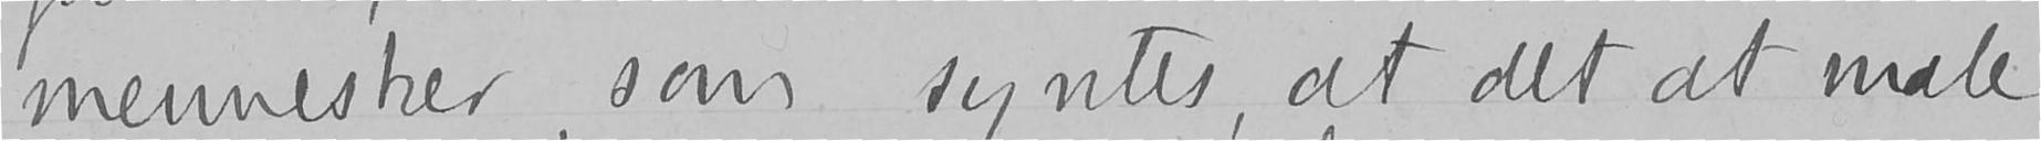mennesker, som syntes, at det at male
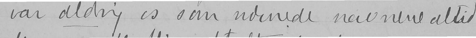var aldrig os som nævnede navnene altid
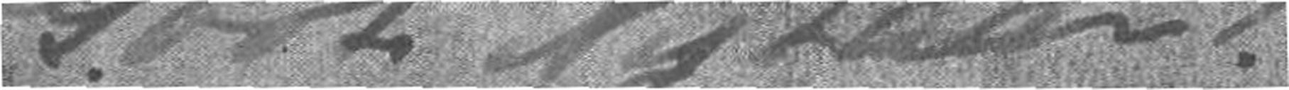Godt Nytaar!
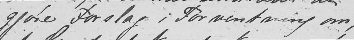gjøre Forslag i Forventning om
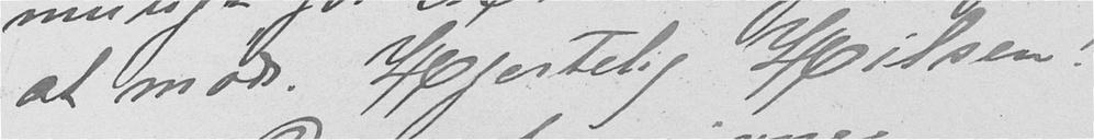at møde. Hjertelig Hilsen!
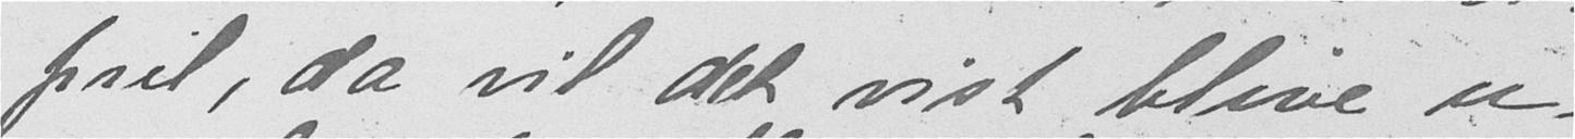pril, da vil det vist blive u-
